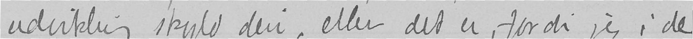udvikling skyld deri, eller det er, fordi jeg i de
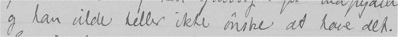og han vilde heller ikke ønske at have det.
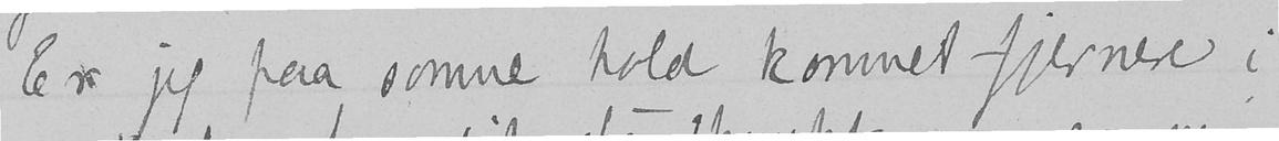Er jeg paa somme hold kommet fjernere i
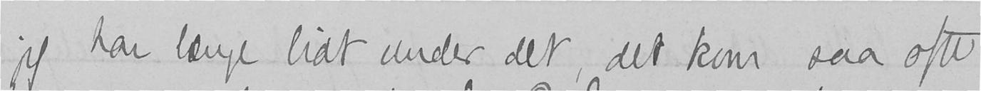jeg har længe lidt under det, det kom saa ofte
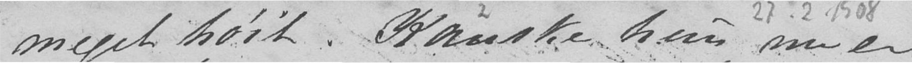meget høit. Kanske hun nu er
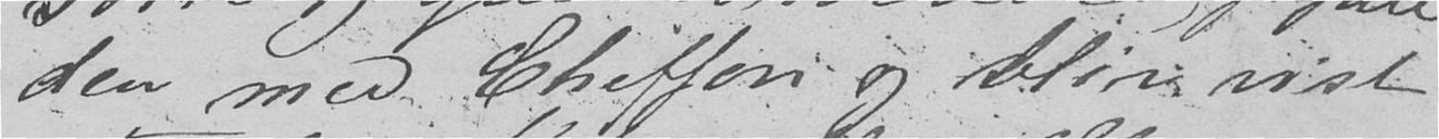den med Chiffon og blir vist
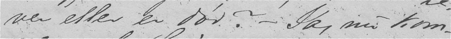ver eller er død? - Ja, nu kom-
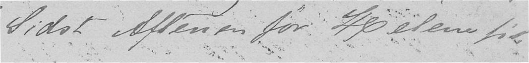Sidst Aftenen før Helene fik
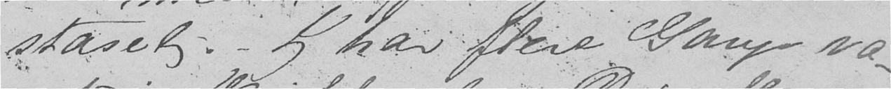staselig. - Jeg har flere Gange væ-
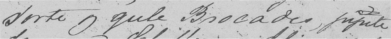sorte og gule Brocades, pynte
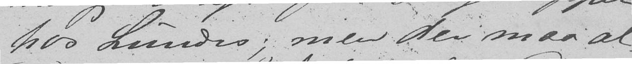hos Lundes; men der maa al-
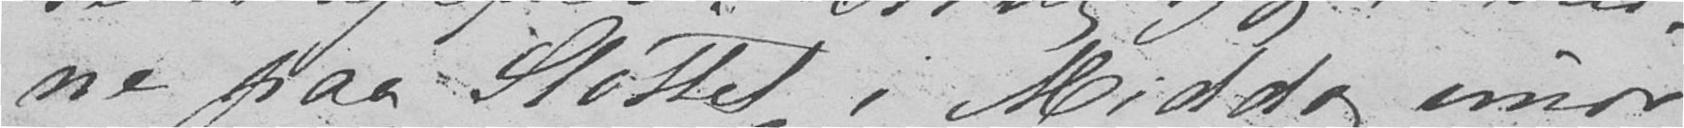ne paa Slottet i Middag imor-
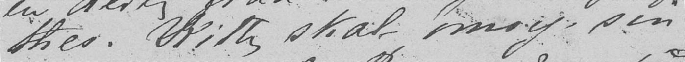thes. Kitty skal omsye sin
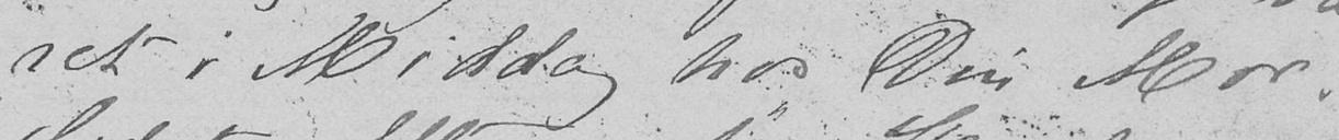ret i Middag hos Din Mor.
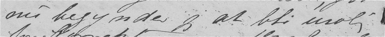nu begynder jeg at bli urolig
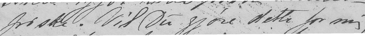friske. Vil Du gjøre dette for mig,
